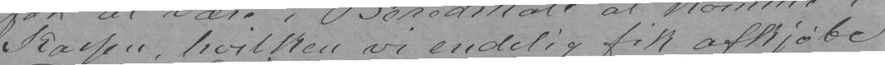Kassen, hvilken vi endelig fik afkjøbe
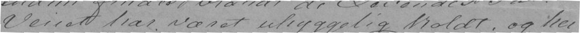Veiret har været uhyggelig koldt, og her
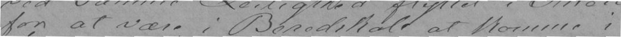for at være i Beredskab at komme i
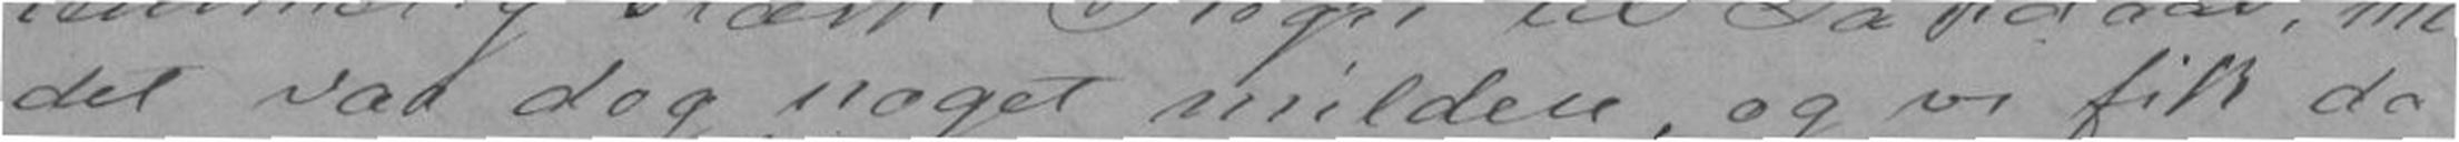det var dog noget mildere, og vi fik da
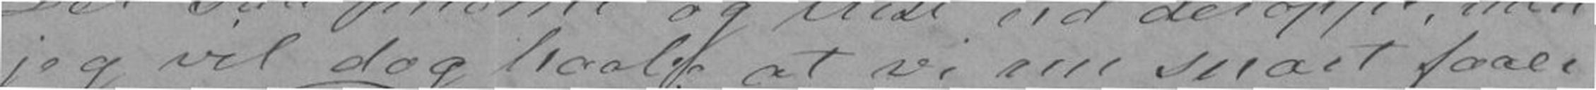jeg vil dog haabe at vi nu snart faaer
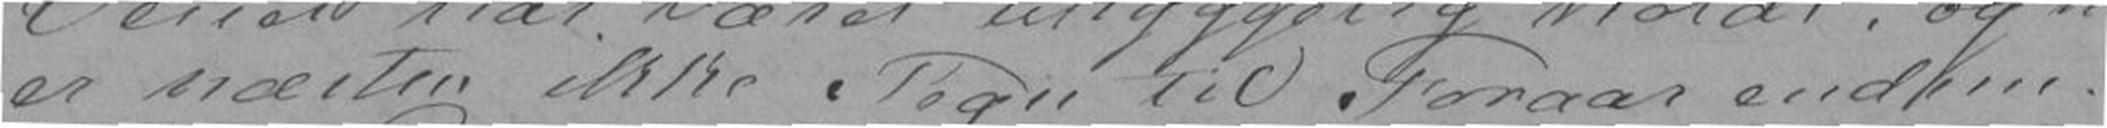er næsten ikke Tegn til Foraar endnu.
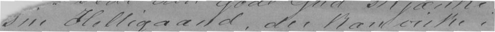in Helligaand, der kan virke i
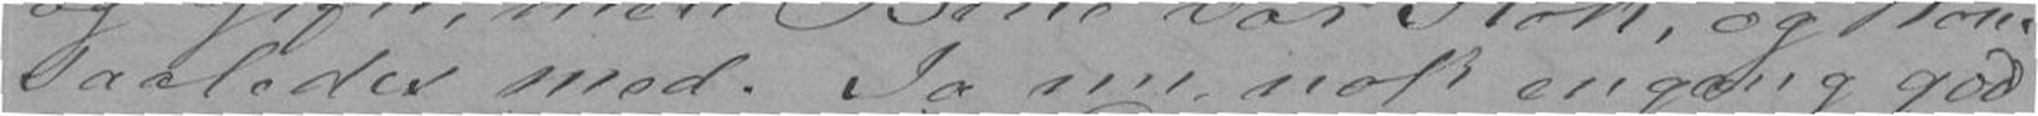saaledes med. Ja nu nok engang god
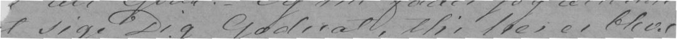t sige Dig Godnat, thi her er blevet
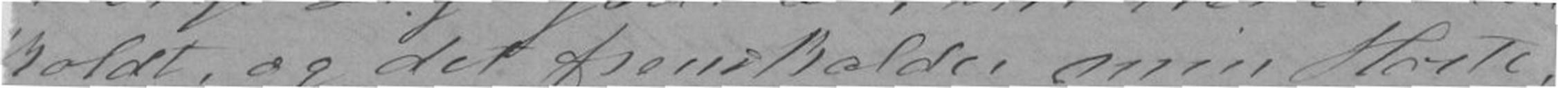koldt, og det fremkalder min Hoste,
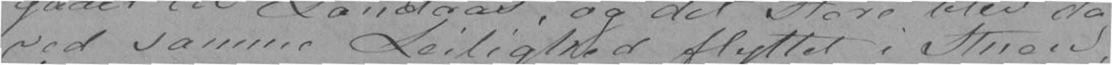ved samme Leilighed flyttet i Stuen,
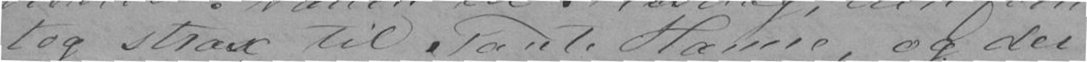tog strax til Tante Hanne, og der
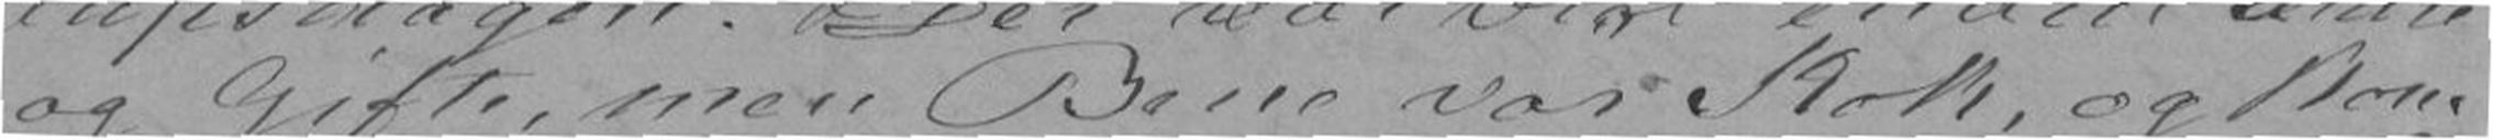og Gifte, men Bene var Kok, og kom
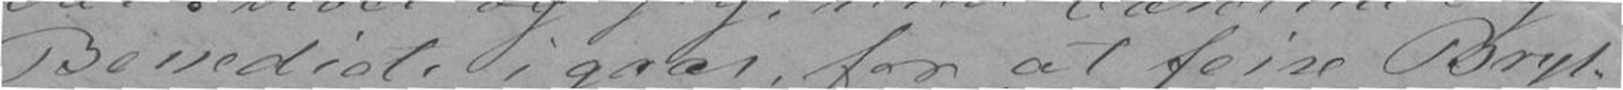Benedicte i gaar, for at feire Bryl-
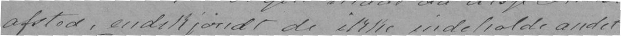afsted, endskjøndt de ikke indeholde andet
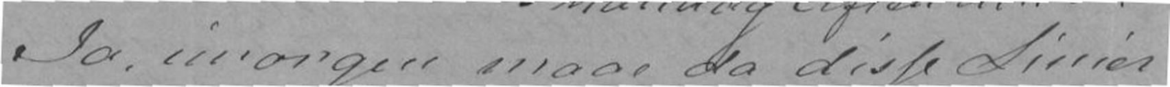Ja imorgen maae da disse Linier
